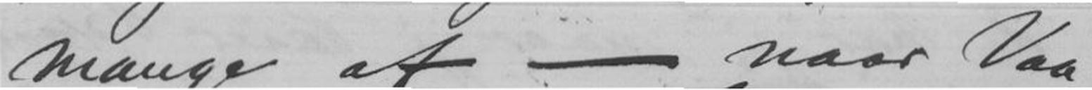mange af --- naar Vaa-
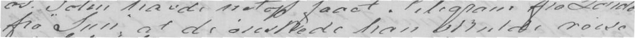fra Sun, at de ønskede han skulde reise
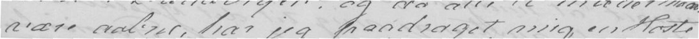være aabne, har jeg paadraget mig en Hoste
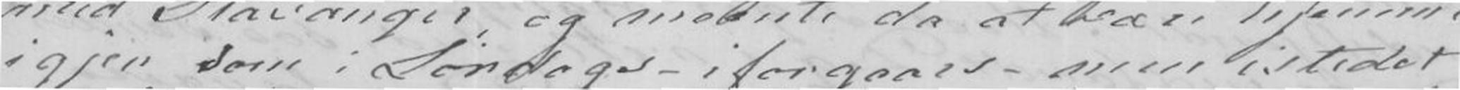igjen som i Lørdags - iforgaars - men istedet
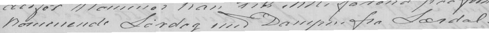kommende Lørdag med Dampen fra Lærdal.
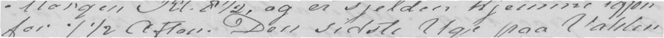for 7½ Aften. Den sidste Uge paa Vahlen
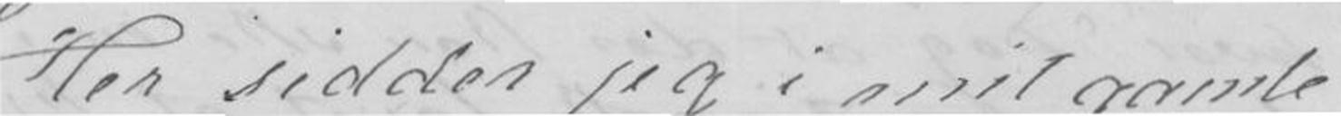Her sidder jeg i mit gamle
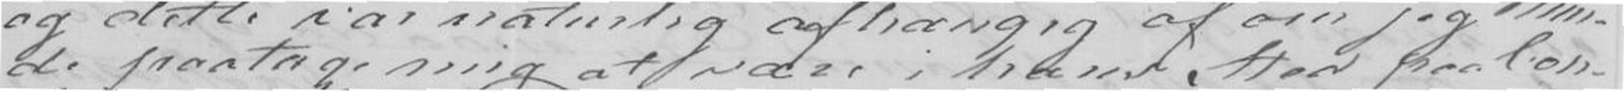de paatage mig at være i hans Sted paa Con-
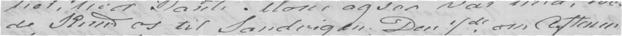de Knud os til Sandvigen. Den 7de om Aftenen
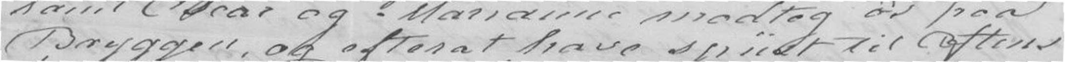Bryggen, og efterat have spiist til Aftens
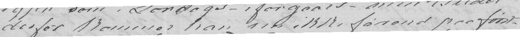derfør kommer han nu ikke førend paa først-
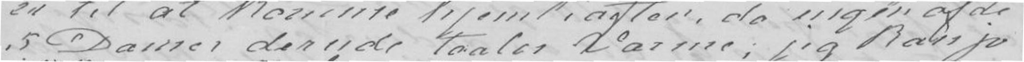5 Damer derude taaler Varme; jeg kan jo
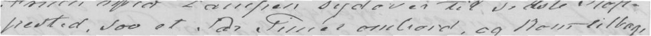pested, saa et Par Timer ombord, og kom Tilbage
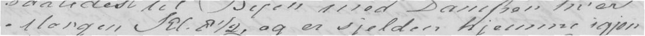Morgen Kl.8½, og er sjelden hjemme igjen
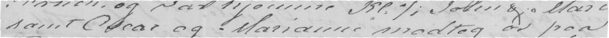samt Oscar og Marianne modtog os paa
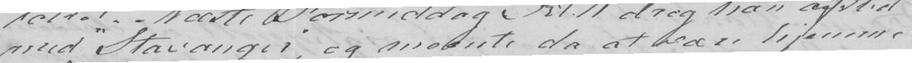med Stavanger og meente da at være hjemme
